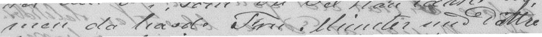men da havde Fru Münster med Døttre
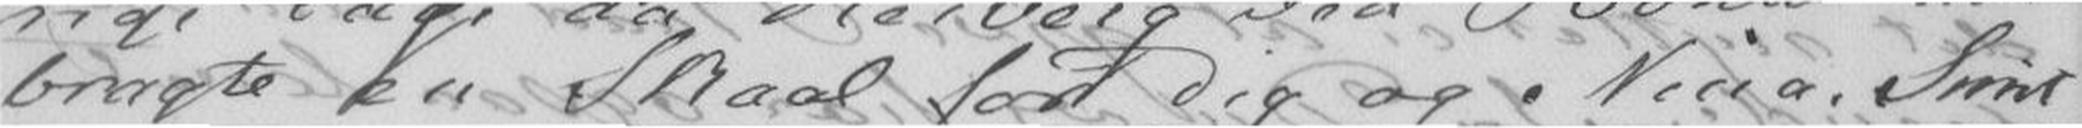bragte en Skaal for Dig og Nina. Smit
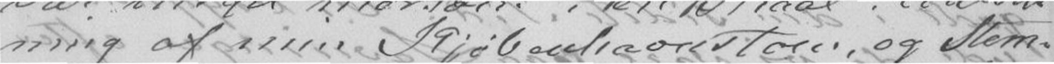ning af min Kjøbenhavnstour, og Stem-
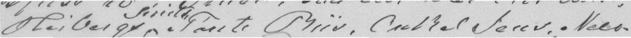Heibergs Smith Tante Riis, Onkel Jens. Neer-
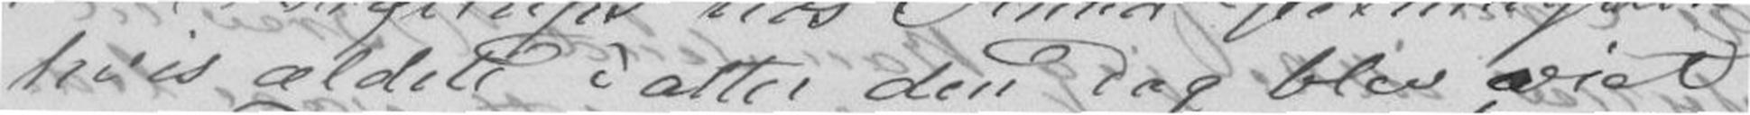hvis ældste Datter den Dag blev viet
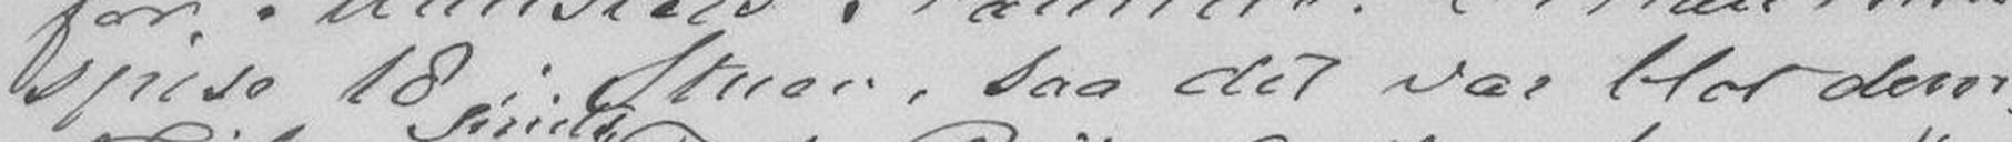spise 18 i Stuen, saa det var blot dem
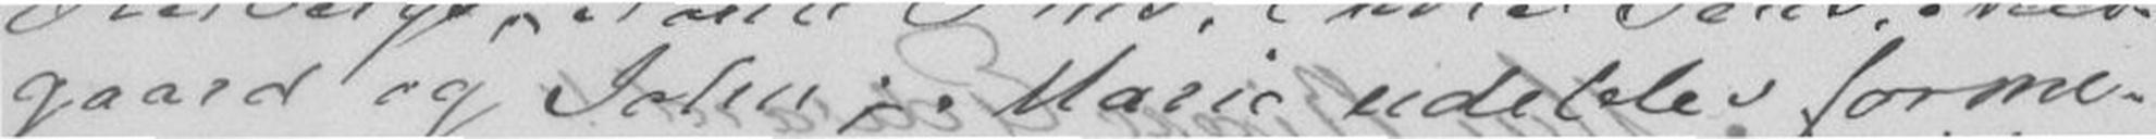gaard og John; Marie udeblev forme-
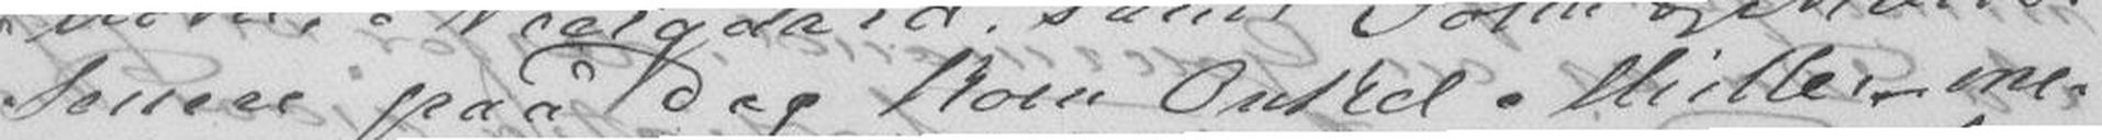Senere paa Dag kom Onkel Müller - me-
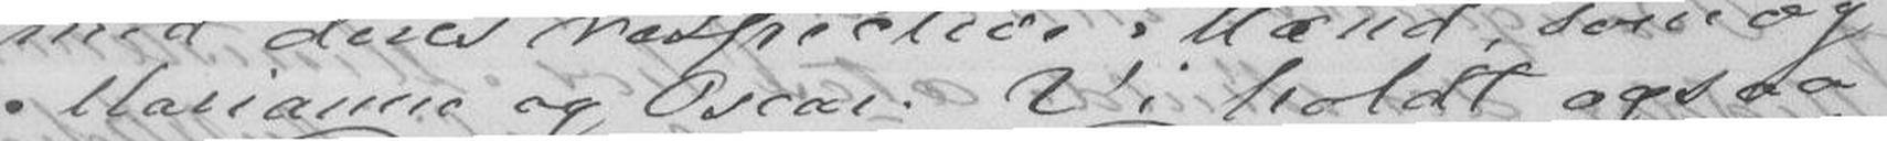Marianne og Oscar. Vi holdt ogsaa
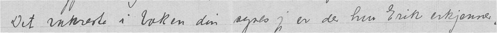Det vakreste i boken din synes jeg er der hvor Erik erkjenner,
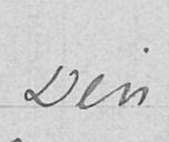Din
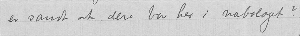er sandt at dere bor her i nabolaget?
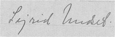Sigrid Undset.
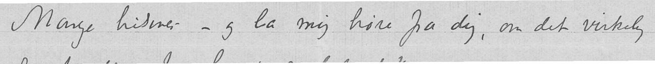Mange hilsener – og la mig høre fra dig, om det virkelig
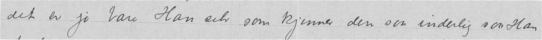det er jo bare Han selv som kjenner den saa inderlig saa Han
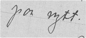paa nytt.
